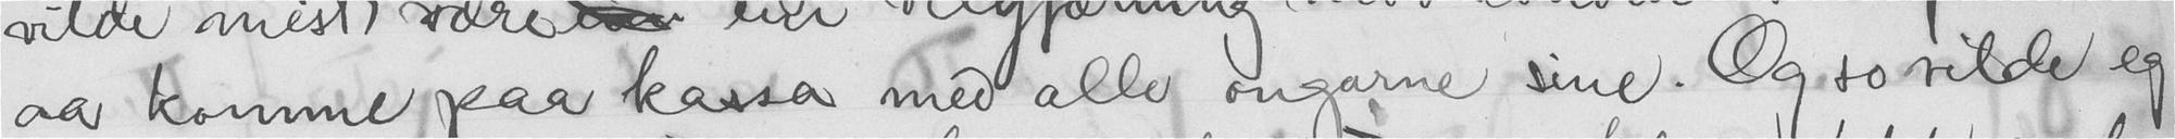aa komme paa kass med alle ongarne sine. Og so vilde eg
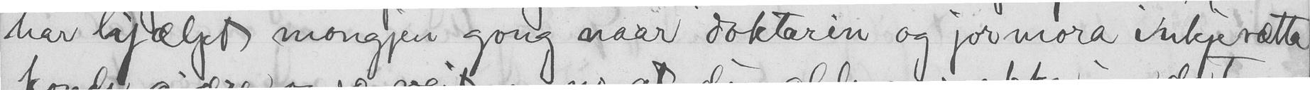har hjælpt mongjen gong naar doktorin og jormora inkje rætte
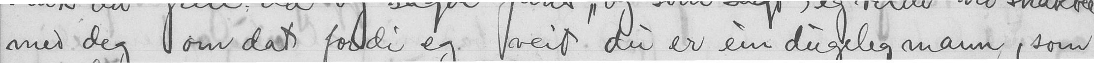med dig om dat fordi eg veit du er ein dugeleg mann, som
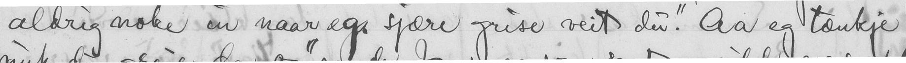aldrig noke in naar eg sjære grise veit du". Aa eg tænkje
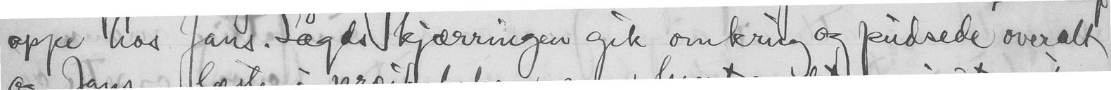oppe hos Jans. Sagds kjærringen gik omkring og pudsede overalt
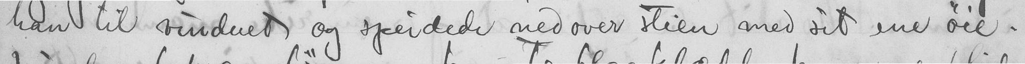han til vinduet og speidede nedover stien med sit ene øie.
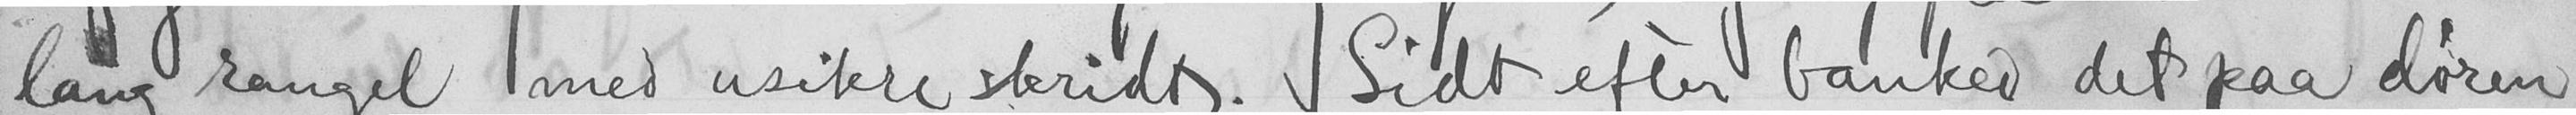lang rangel med usikre skridt. Sidt efter banked det paa døren
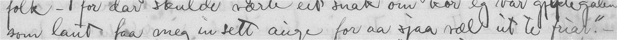som laut faa mig insett auge for aa "sjaa væl ut te friar." -
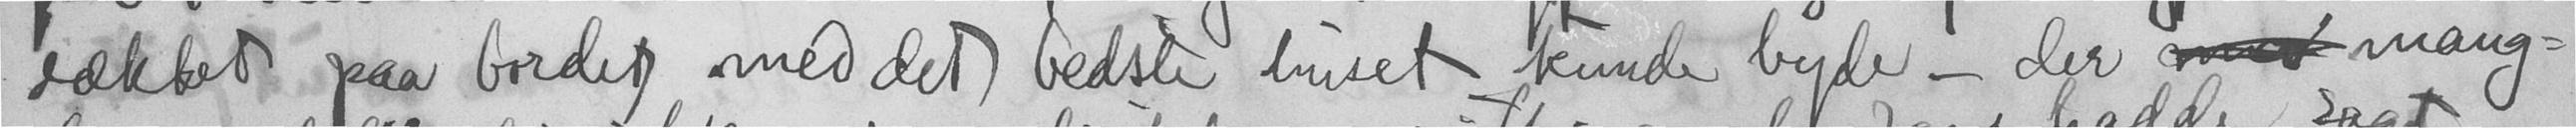dækket paa bordet med det bedste huset kunde byde - der  mang-
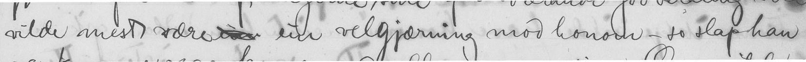vilde mest være  ein velgjærning mod honom - so slap han
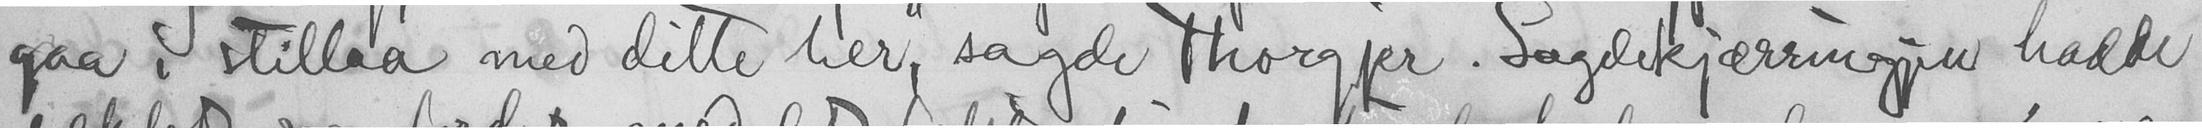gaa i stillaa med dette her," sagde Thorgjer. Sagde kjærringen hadde
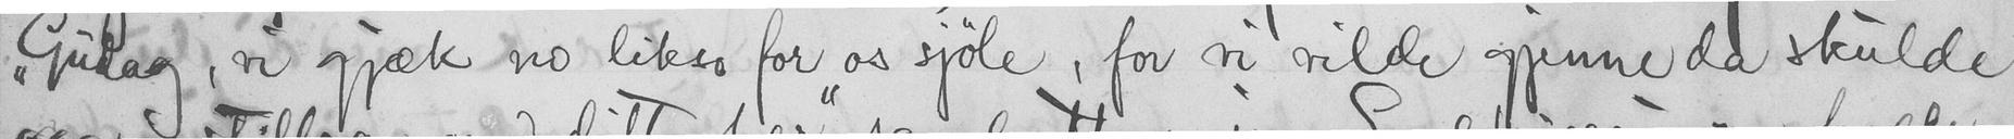"Gudag, vi gjæk no likes for os sjøle, for vi vilde gjenne da skulde
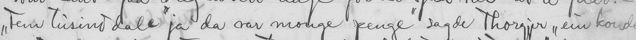"Fem tusind dale" ja da var monge penge" sagde Thorgjer "ein konde
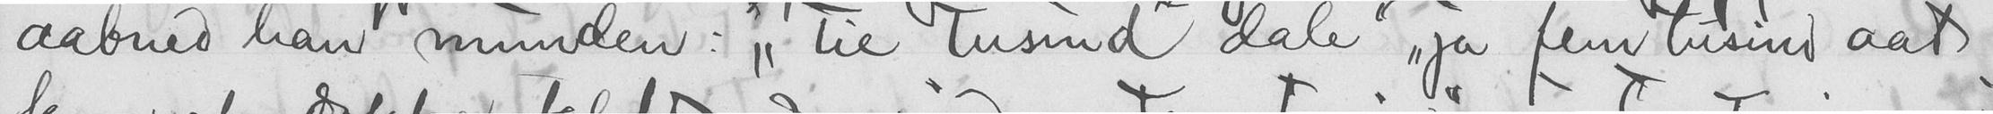aabned han munden: "tie tusind dale" "ja fem tusind aat
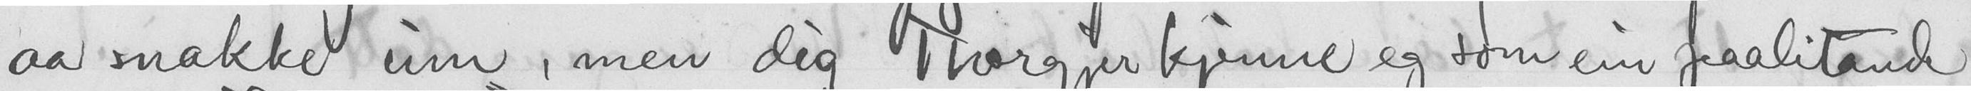aa snakke um, men dig Thorgjer kjenne eg som ein paalitande
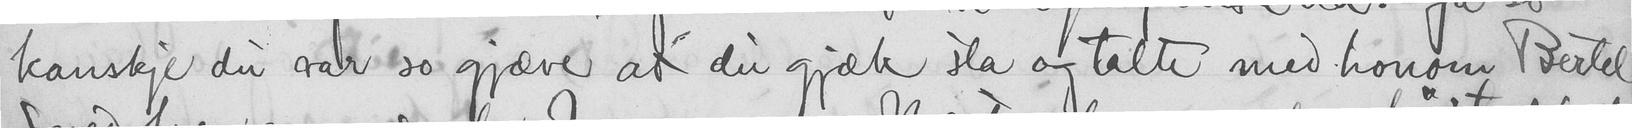kanskje du var so gjæve at du gjæk sta og talte med honom Bertel
